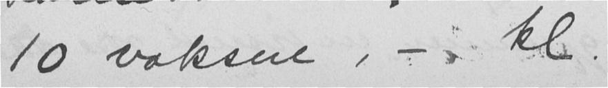10 vokse, - kl.
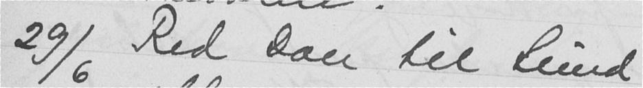29/6 Red Don til Sund
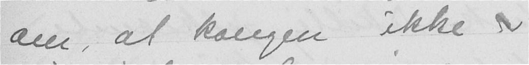om, at kongen ikke er
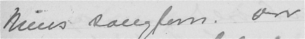Mens sangforn. var
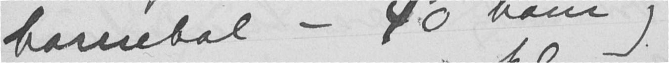barnebal - 40 barn og
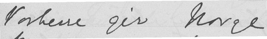Vorhere gir Norge
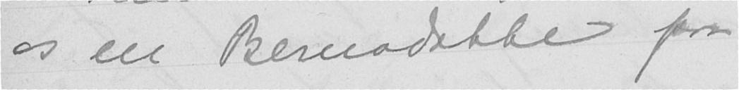os en Bernadotte paa
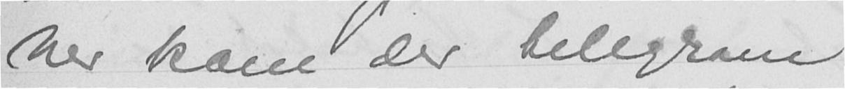her kom der telegram
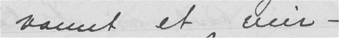varmt et veir -
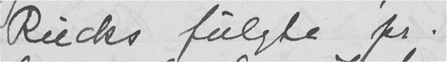Riecks fulgte pr.
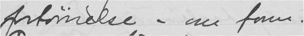fortørnelse - om form.
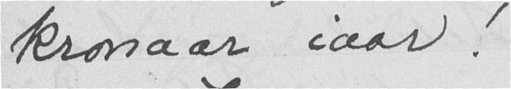kronaar iaar!
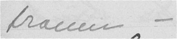tronen -
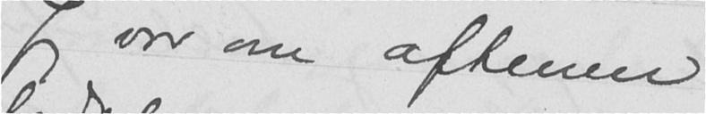Jeg var om aftenen
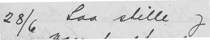28/6 Saa stille og
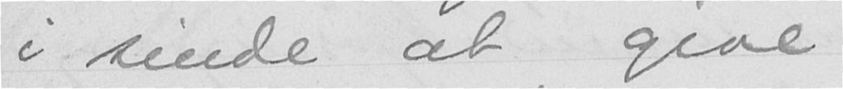i sinde at give
